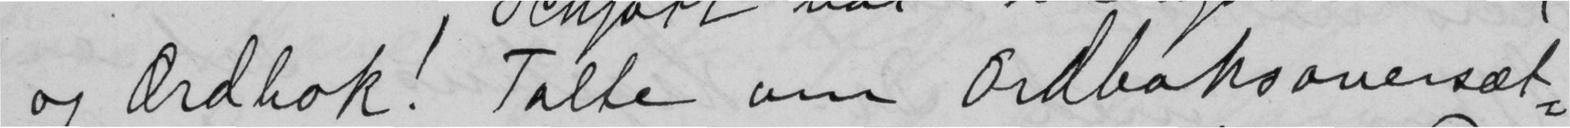og Ordbok! Talte om Ordboksoversæt-
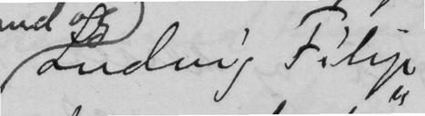Ludwig Filip
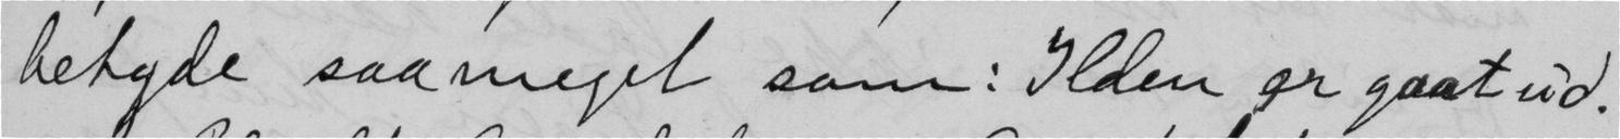betyde saameget som: Ilden er gaat ud.
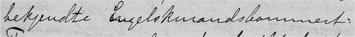bekjendte Engelskmandsbommert:
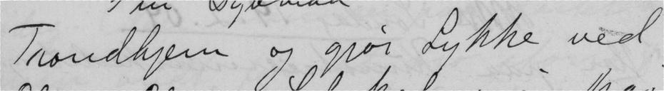Trondhjem og gjør Lykke ved
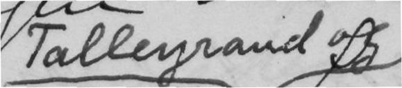Talleyrand og
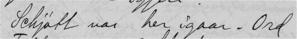Schjøtt var her igaar. Ord
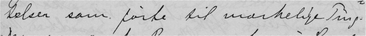telser som førte til mærkelige Ting.
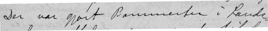Der var gjort Bommerter i Lands-
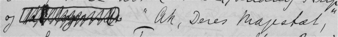og . "Ak. Deres Magestæt,"
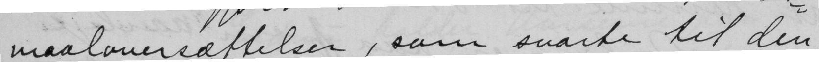maaloversættelser, som svarte til den
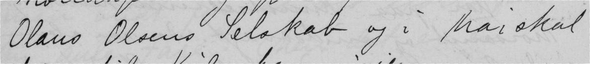Olaus Olsens Selskab og i Mai skal
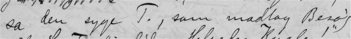sa den syge T., som modtog Besøg
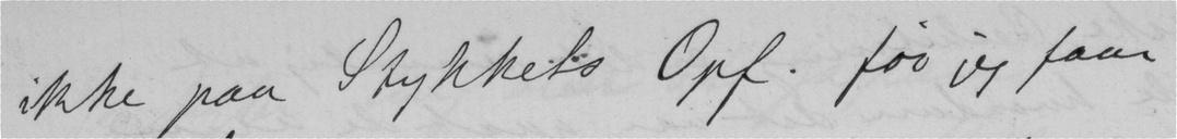ikke paa Stykkets Opf. før jeg faar
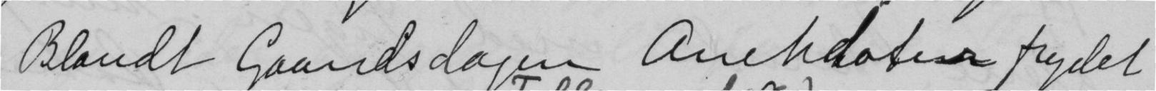Blandt Gaardsdagen Anekdoter frydet
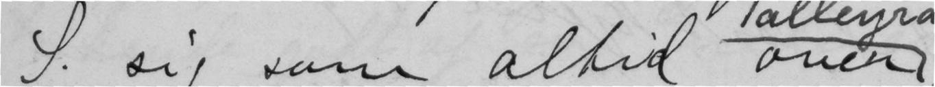S. sig som altid over
